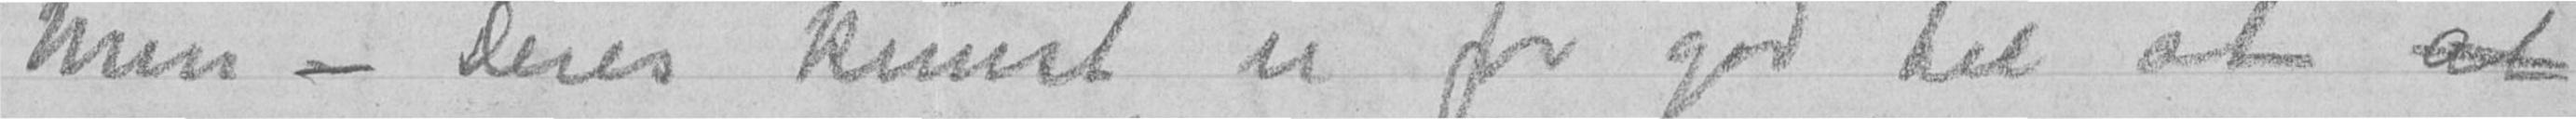Men - Deres kunst er for god til at at
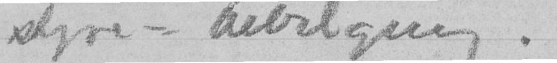styre-bevilgning.
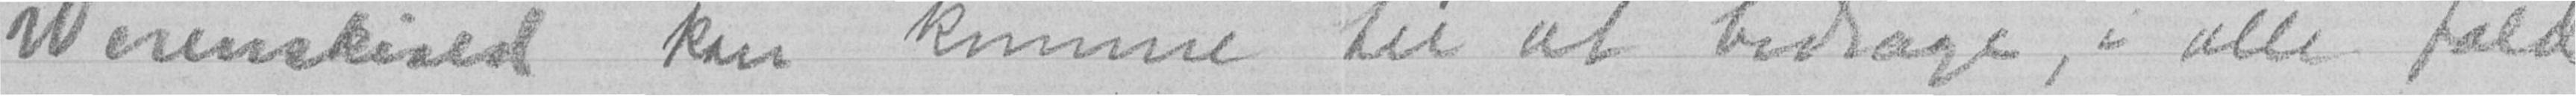Werenskiold kan komme til at bidrage, i alle fald
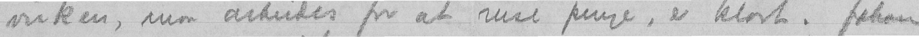virken, maa arbeides for at reise penge, er klart. Johan
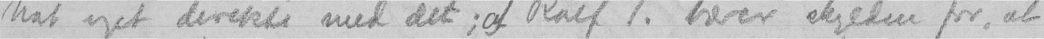hat noget direkte med det; at Rolf T. har skylden for, at
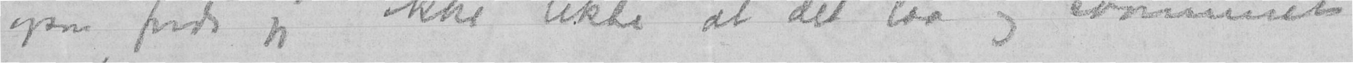ogsaa fordi jeg ikke likte at det laa og svømmet
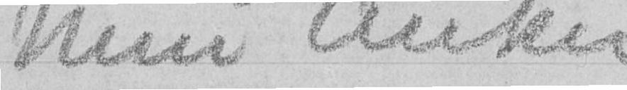Nini Anker
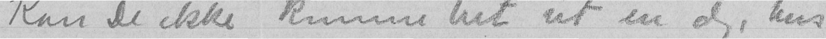Kan De ikke komme hit ut en dag, hvis
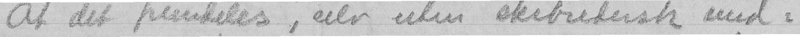At det fremdeles, selv uten skibredersk med-
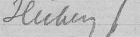Heiberg).
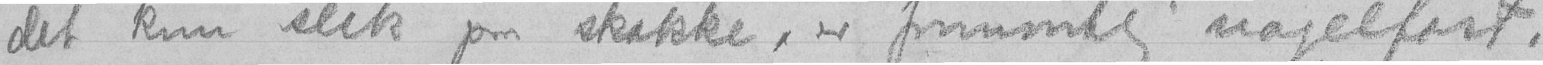det kom slik paa skakke, er formontlig nagelfast.
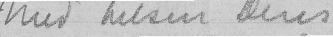Med hilsen Deres
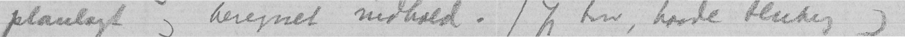planlagt og beregnet indhold. (Jeg tror, baade Heiberg og
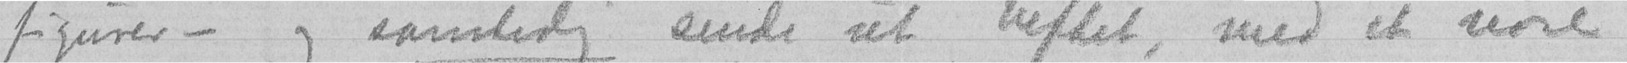figurer - og samtidig sende ut heftet, med et nøie
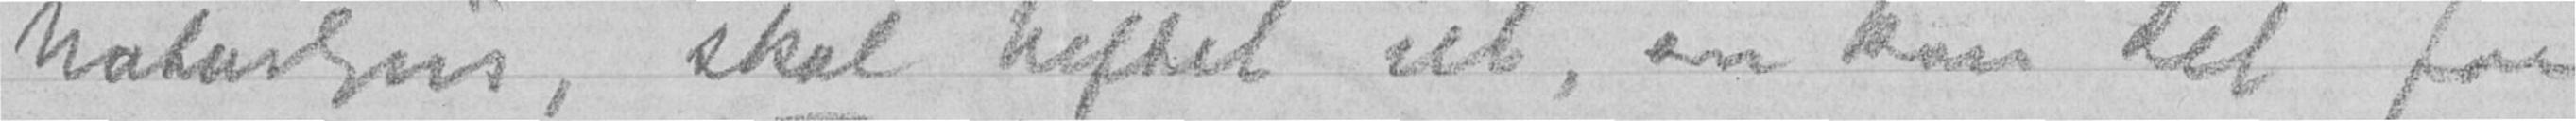Naturligvis, skal heftet ut, saa kan det faa
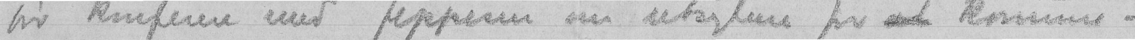vil konferere med Jeppesen om utsigten for et kommune-
